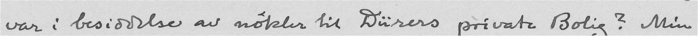var i besiddelse av nøkler til Dürers private Bolig? Min
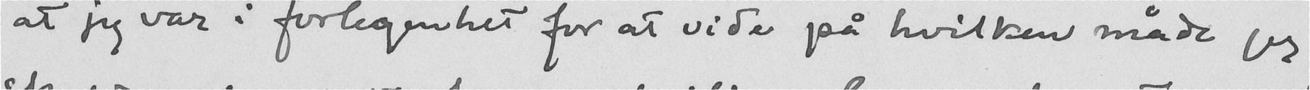at jeg var i forlegenhet for at vide på hvilken måde jeg
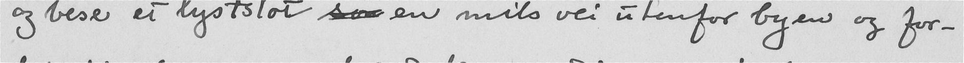og bese et lystslot som en mils vei utenfor byen og for-
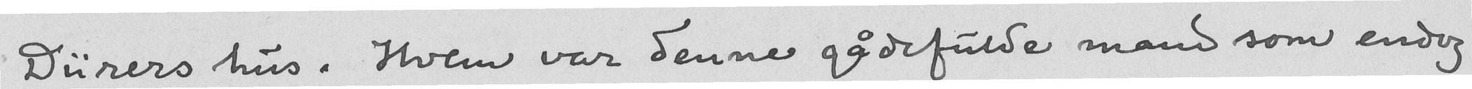Dürers hus. Hvem var denne gådefulde mand som endog
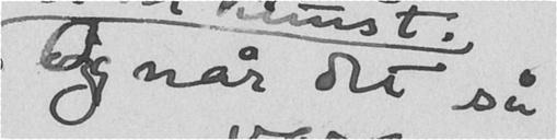Og når det så
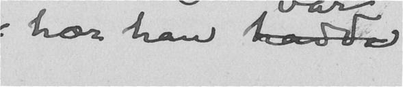hær han hadde
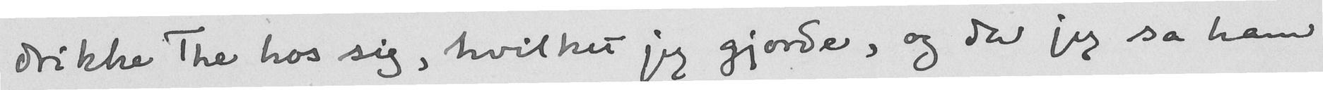drikke The hos sig, hvilket jeg gjorde, og da jeg sa ham
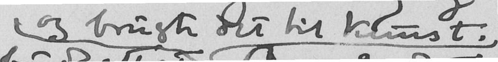og brugte det til kunst.
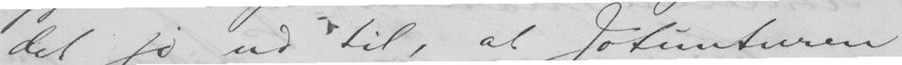det jo ud til, at Jotunturen
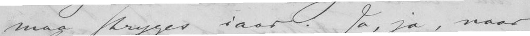maa stryges iaar. Ja, ja, naar
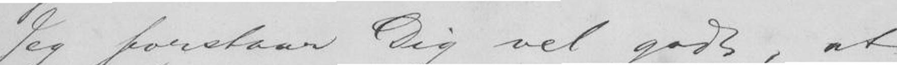Jeg forstaar Dig vel godt, at
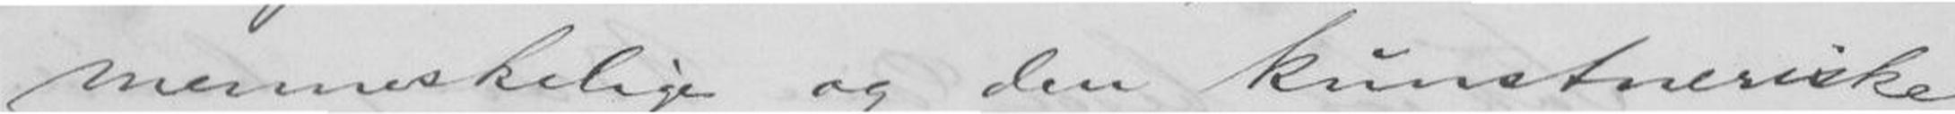menneskelige og den kunstneriske,
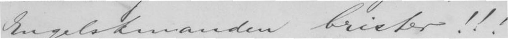Engelskmanden brister!!!
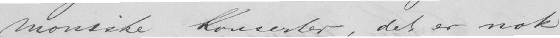moniske Konserter, det er nok
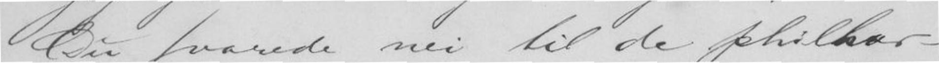Du svarede nei til de philhar-
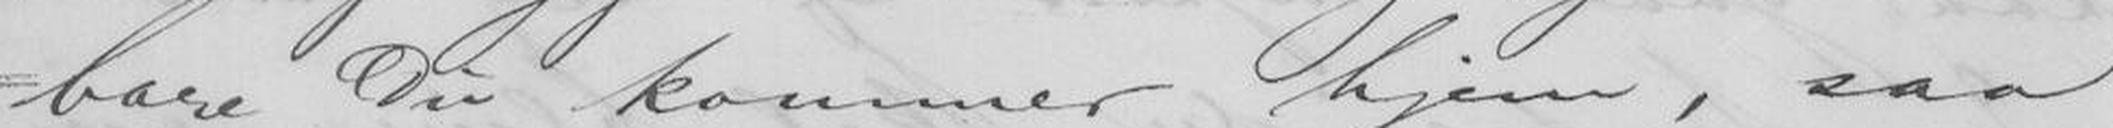bare Du kommer hjem, saa
